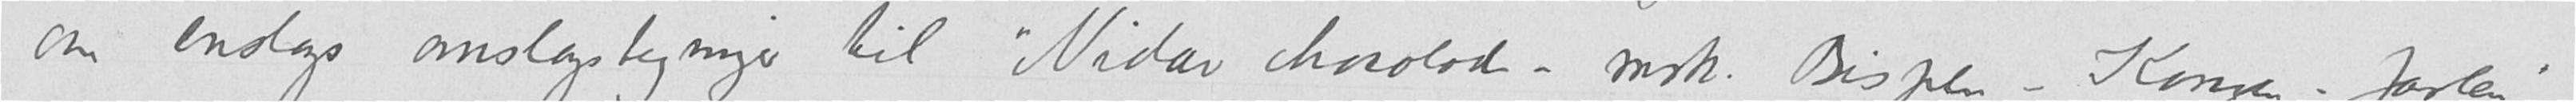om enslags omslagstegninger til "Nidar chocolade – mrk. Bispen – Kongen – Jarlen"
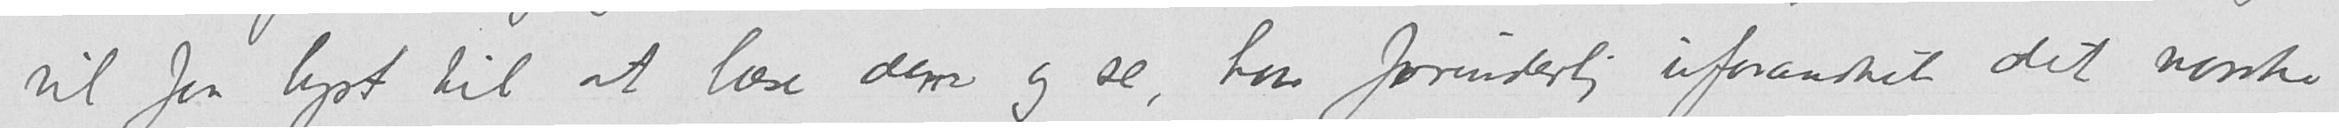vil faa lyst til at læse dem og se, hvor forunderlig uforandret det norske
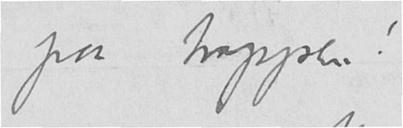paa trappen!
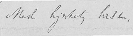Med hjertelig hilsen,
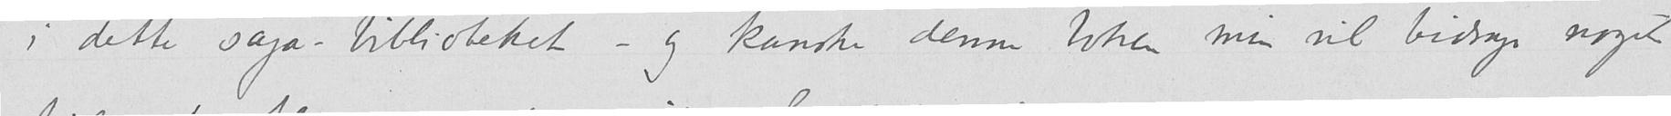i dette saga-biblioteket – og kanske denne boken min vil bidrage noget
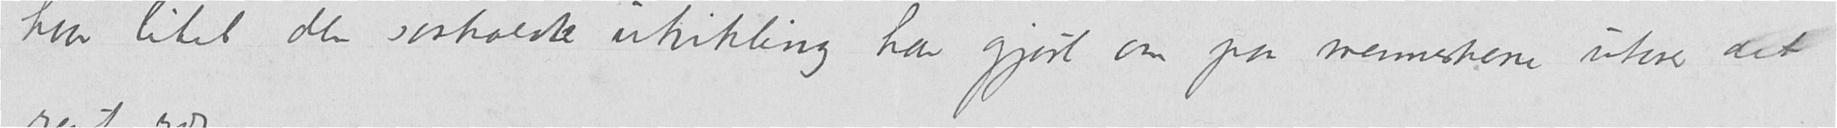hvor litet den saakaldte utvikling har gjort om paa menneskene utover det
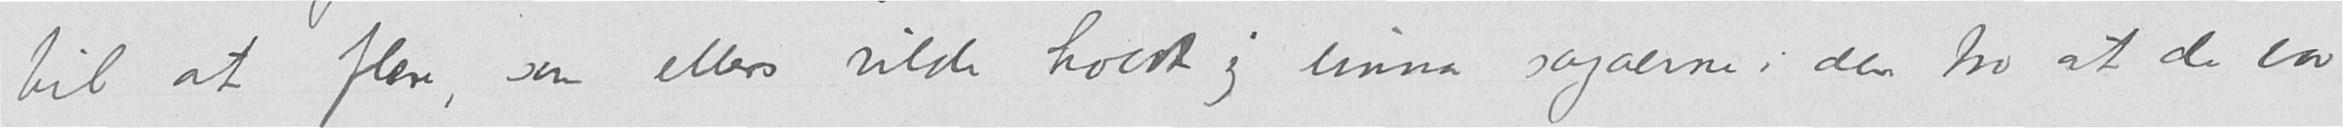til at flere, som ellers vilde holdt sig unna sagaerne i den tro at de var
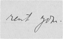rent ydtre.
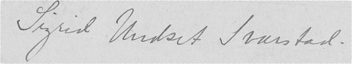Sigrid Undset Svarstad.
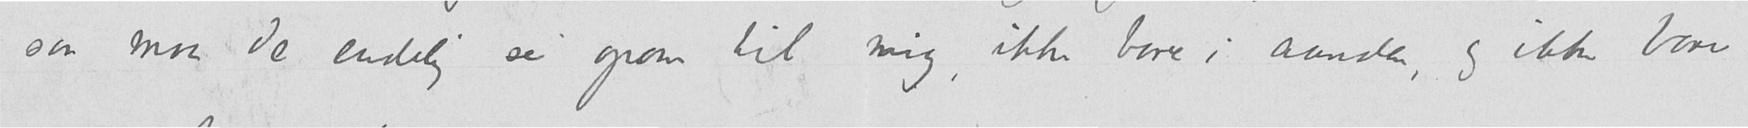saa maa De endelig se opom til mig, ikke bare i aanden, og ikke bare
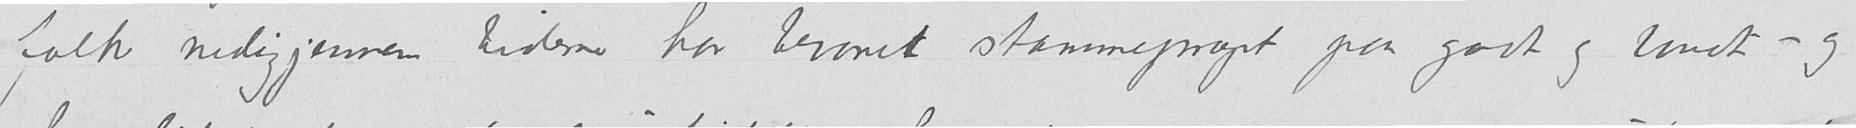folk nedigjennem tiderne har bevaret stammepreget paa godt og vondt – og
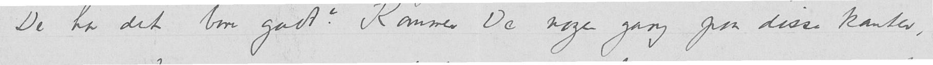De har det bare godt? Kommer De nogen gang paa disse kanter,
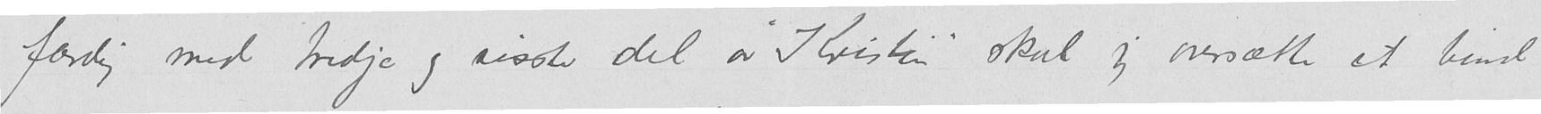færdig med tredje og sisste del av Kristin skal jeg oversætte et bind
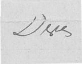Deres
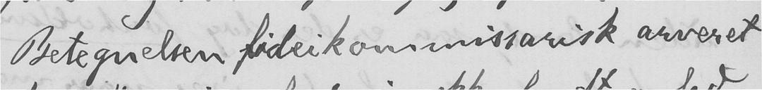Betegnelsen fideikommissarisk arveret
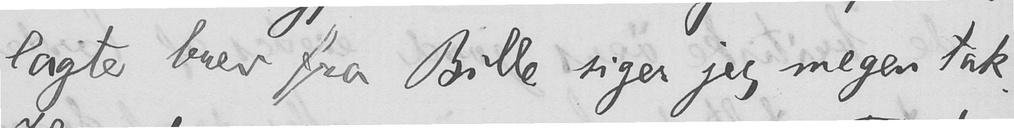lagte brev fra Bille siger jeg megen tak.
